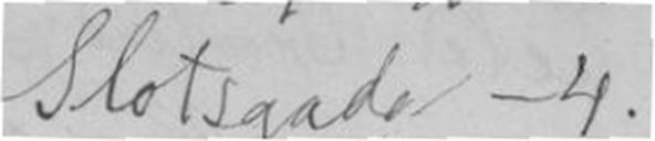Slotsgade -4.
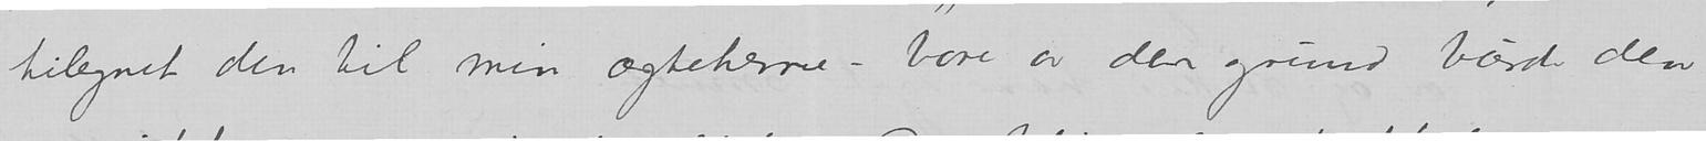tilegnet den til min ægteherre – bare av den grund burde den
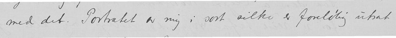med det. Portrætet av mig i sort silke er foreløpig utsat
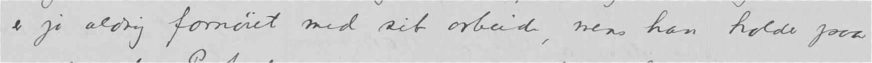er jo aldrig fornøiet med sit arbeide, mens han holder paa
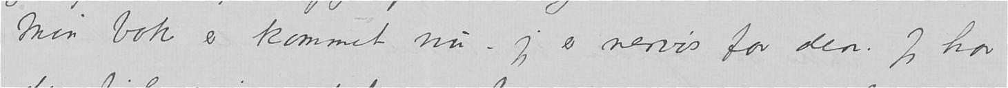Min bok er kommet nu – jeg er nervøs for den. Jeg har
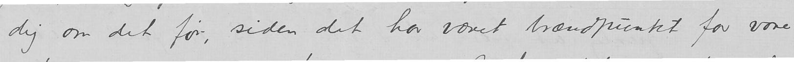dig om det før, siden har været brændpunkt for vore
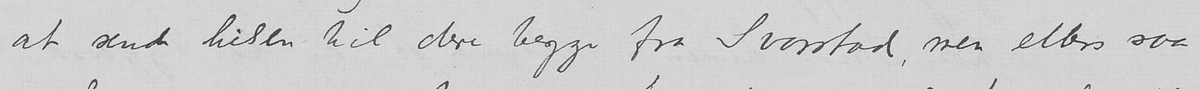at sende hilsen til dere begge fra Svarstad, men ellers saa
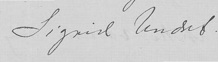Sigrid Undset
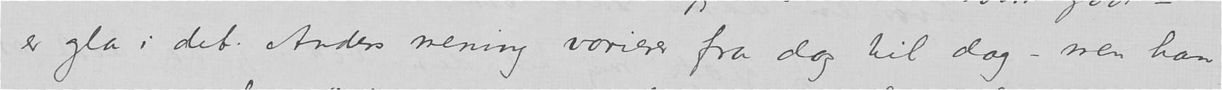er gla i det. Anders {sic} mening varierer fra dag til dag – men han
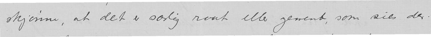skjønne, at det er særlig raat eller gement, som sies der.
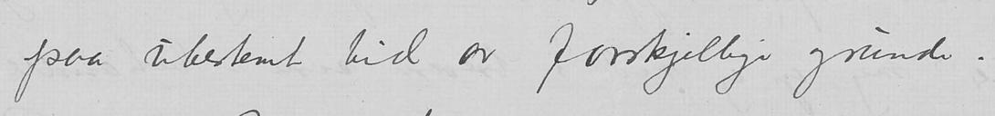paa ubestemt tid av forskjellige grunde.
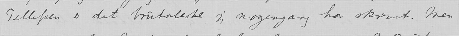Tellefsen er det brutaleste jeg nogengang har skrevet. Men
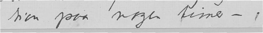tsion paa nogen timer –.
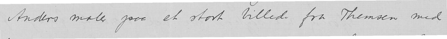Anders maler paa et stort billede fra Themsen med
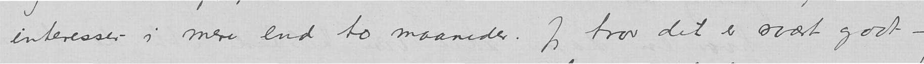interesser i mere end to maaneder. Jeg tror det er svært godt –
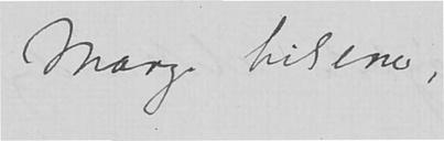Mange hilsener,
